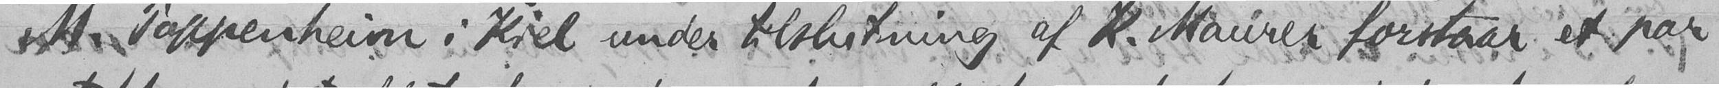M. Pappenheim i Kiel under tilslutning af K. Maurer forstaar et par
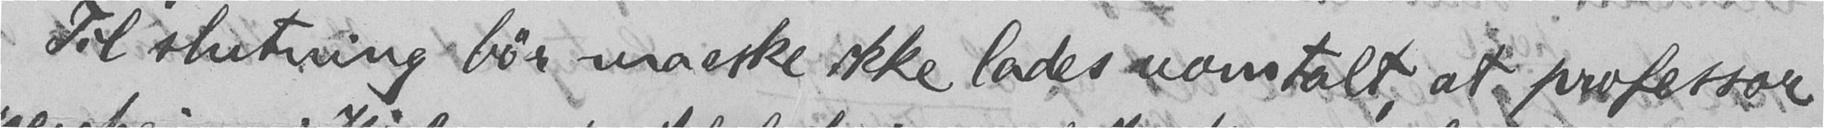Til slutning bør maaske ikke lades uomtalt, at professor
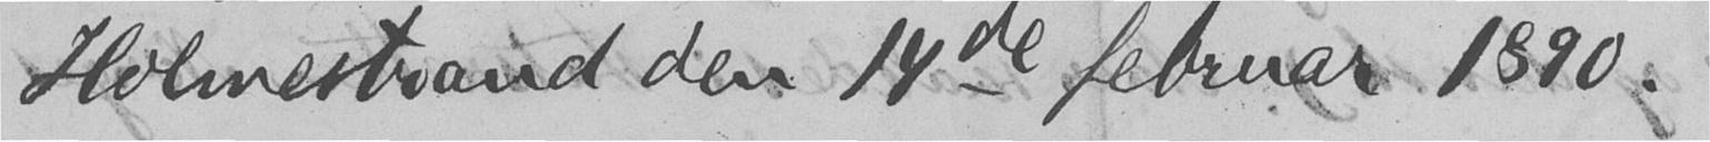Holmestrand den 14de februar 1890.
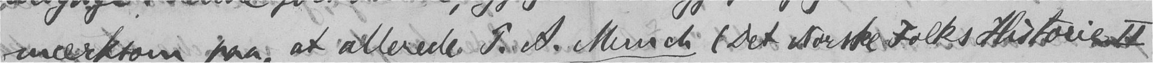mærksom paa, at allerede P. A. Munch (Det Norske Folks Historie II
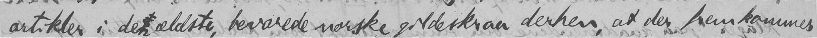artikler i de ældste, bevarede norske gildeskraa derhen, at der fremkommer
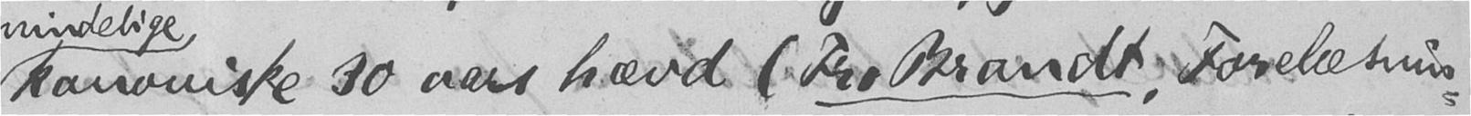kanoniske 30 aars hævd (Fr. Brandt, Forelæsnin-
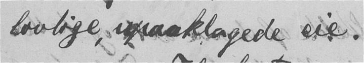lovlige, upaaklagede eie.
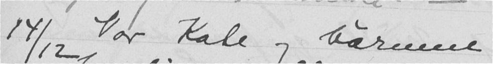14/12 Var Kate og børnene
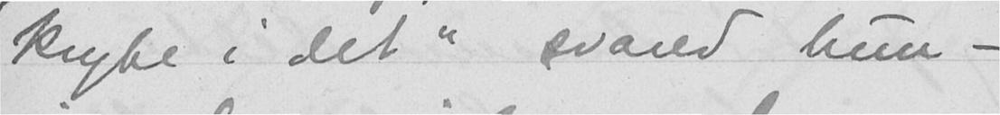krybe i det" svared hun -
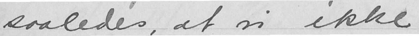saaledes, at vi ikke
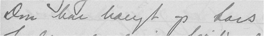Don har hængt os Lars
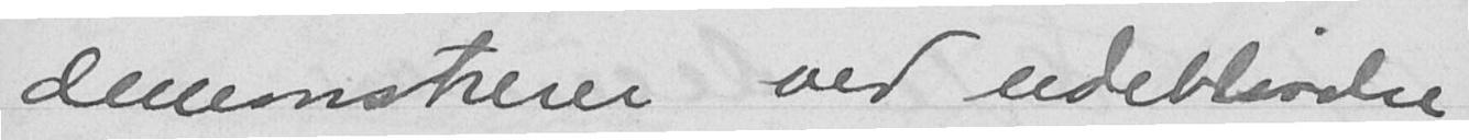demonstrerer ved udeblivelse
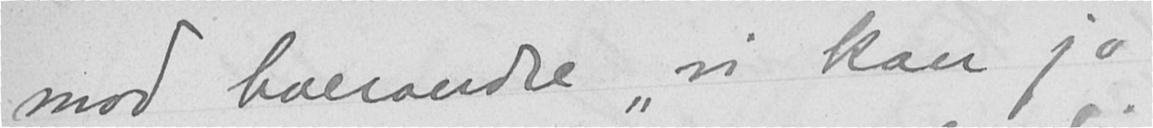mod hverandre "vi kan jo
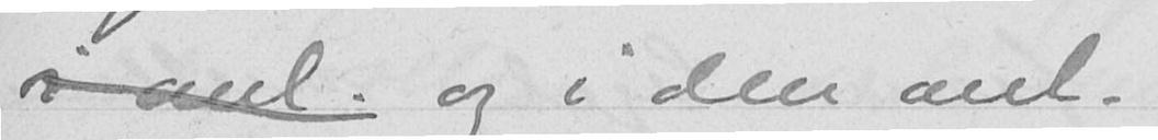i anl. og i den anl.
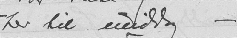her til middag -
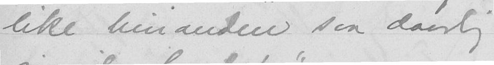like hinanden saa daarlig
